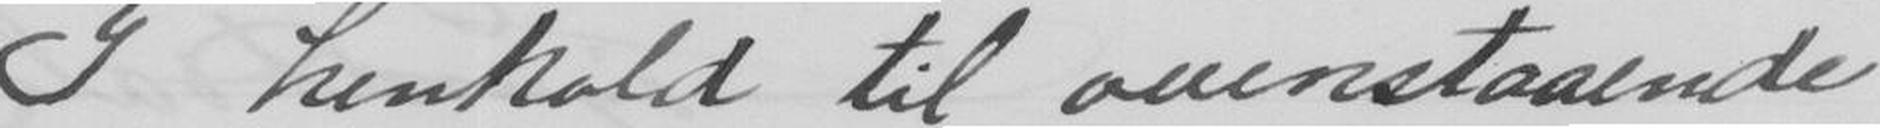I henhold til ovenstaaende
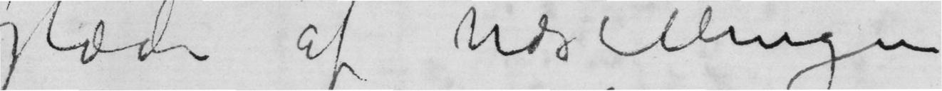Glæde af Udstillingen
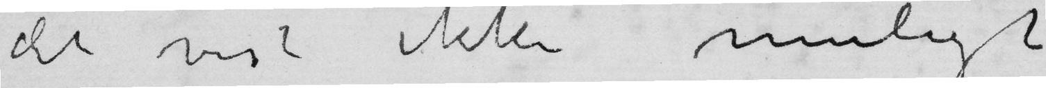det vist ikke muligt
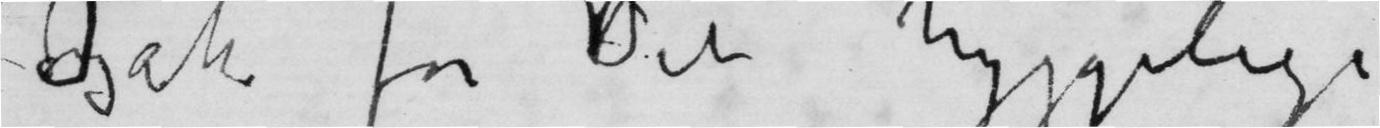Tak for Dit hyggelige
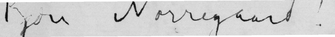Kjære Nørregaard!
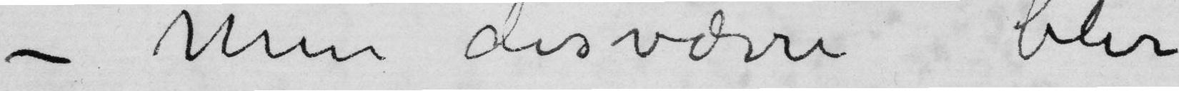– Men desværre blir
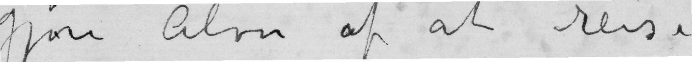gjøre Alvor af at reise
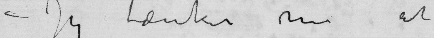– Jeg tænker nu at
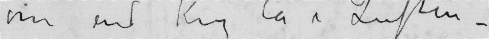om end Krig lå i Luften –
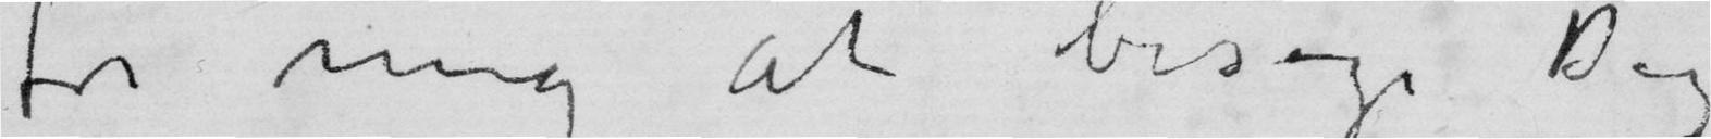for mig at besøge Dig
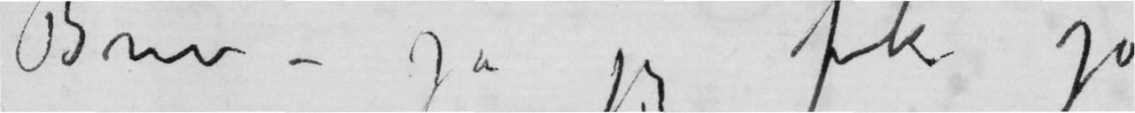Brev – Ja jeg fik jo
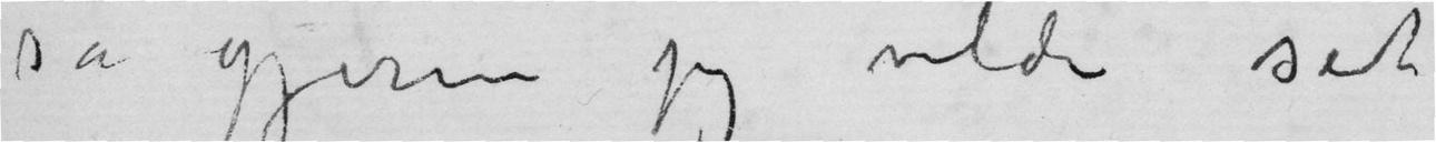så gjerne jeg vilde set
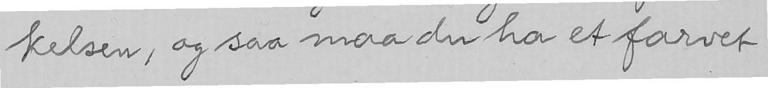kelsen, og saa maa du ha et farvet
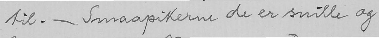til. - Smaapikerne de er snille og
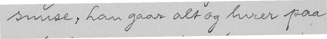snuse, han gaar alt og lurer paa
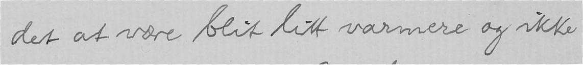det at være blit litt varmere og ikke
